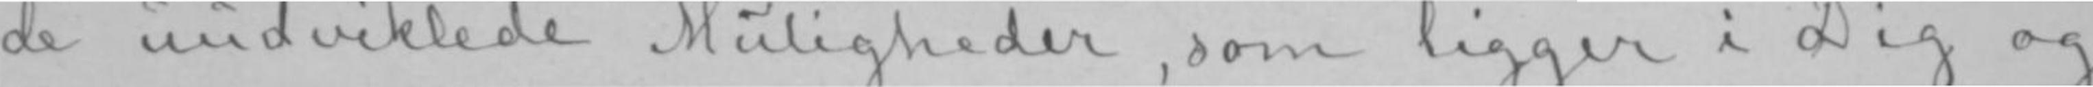de uudviklede Muligheder, som ligger i Dig og
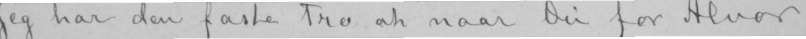Jeg har den faste Tro at naar Du for Alvor
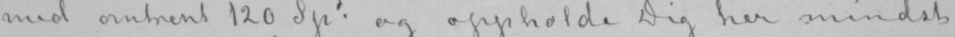med omtrent 120 Spd og oppholde Dig her mindst
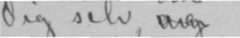Dig selv, og
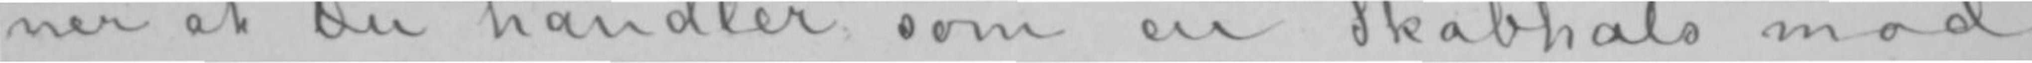ner at Du handler som en Skabhals mod
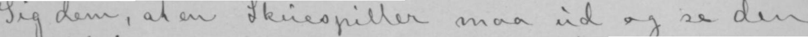Sig dem, at en Skuespiller maa ud og se den
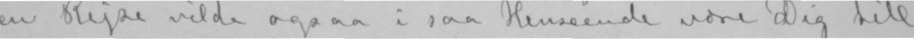en Rejse vilde ogsaa i saa Henseende være Dig till
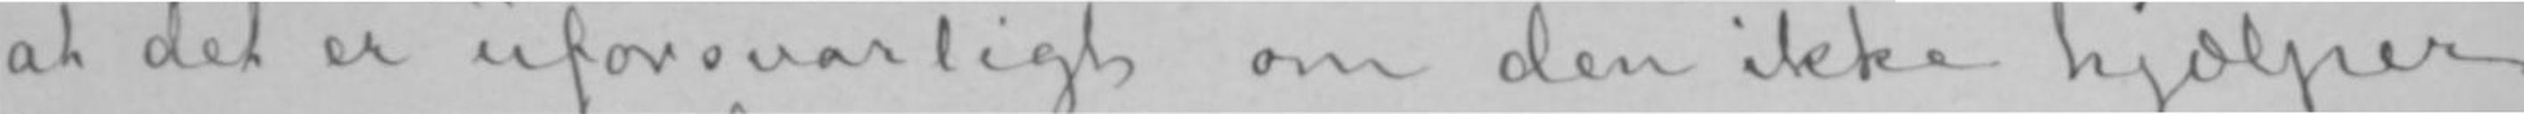at det er uforsvarligt om den ikke hjælper
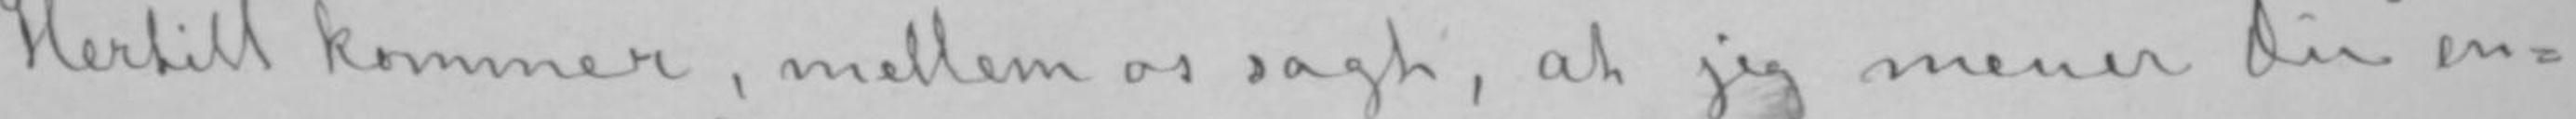Hertill kommer, mellem os sagt, at jeg mener Du en-
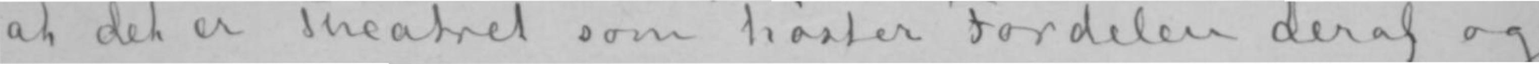at det er Theatret som høster Fordelen deraf og
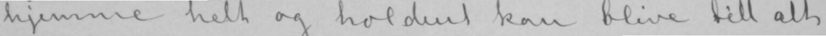hjemme helt og holdent kan blive till alt
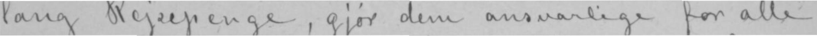lang Rejsepenge, gjør dem ansvarlige for alle
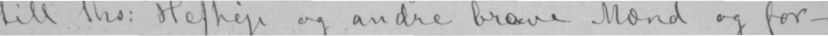till Ths: Heftye og andre bravebre Mænd og for-
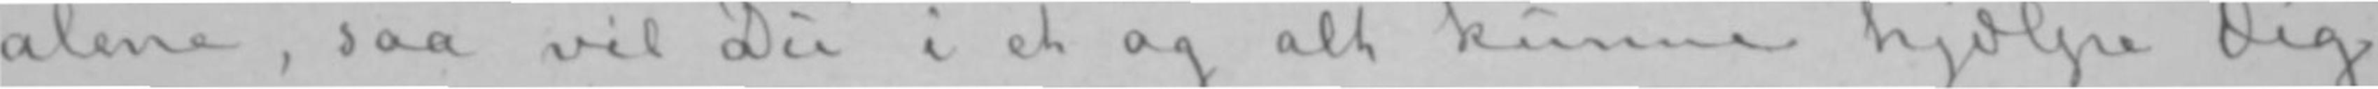alene, saa vil Du i et og alt kunne hjælpe Dig
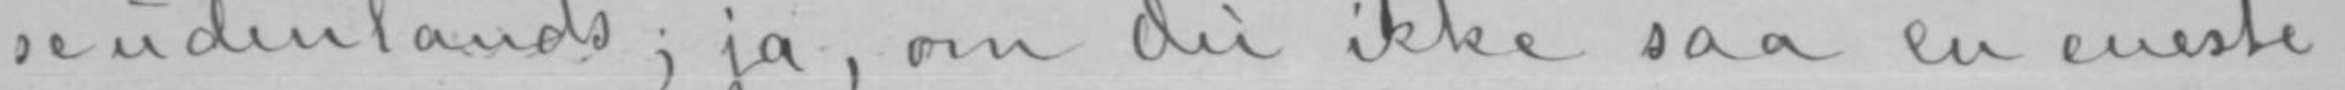se udenlands; ja, om Du ikke saa en eneste,
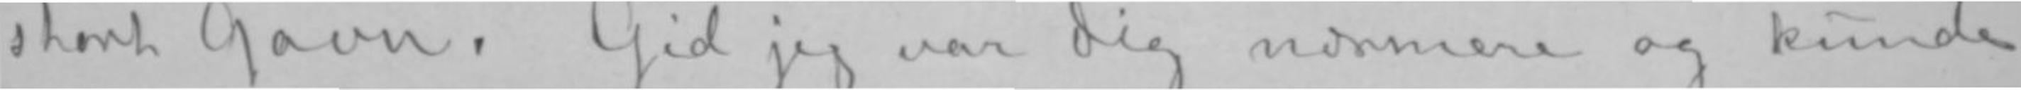stort Gavn. Gid jeg var Dig nærmere og kunde
